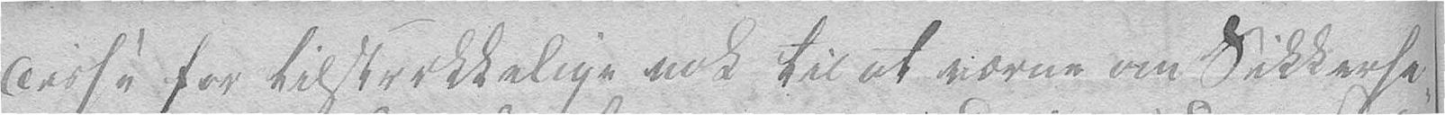disse for tilstrækkelige nok til at værne om Sikkerhe-
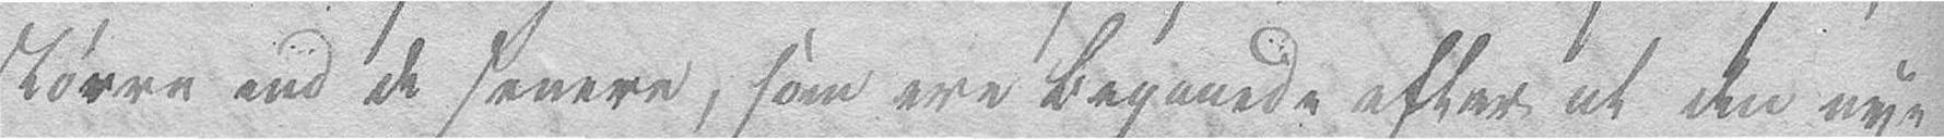større end de senere, som ere begaaede efter at den nye
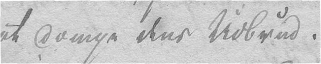at dæmpe dens Udbrud.
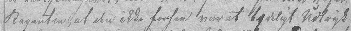Regenten, at den ikke forhen var et tydeligt Udtryk
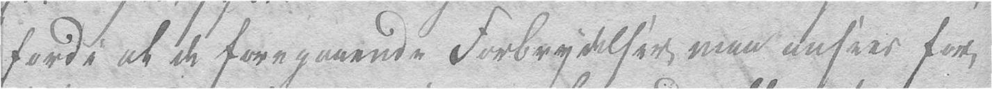fordi at de foregaaende Forbrydelser maa ansees for
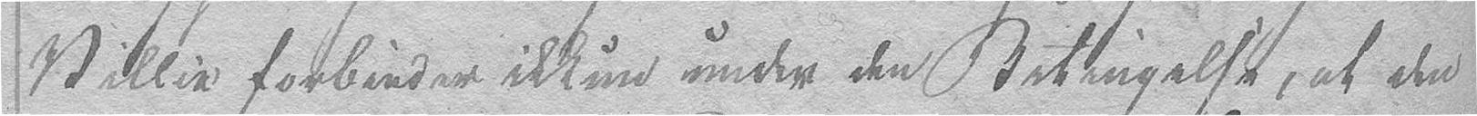Villie forbinder ikkun under den Betingelse, at den
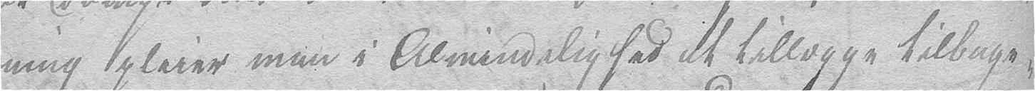ning pleier man i Almindelighed at tillægge tilbage-
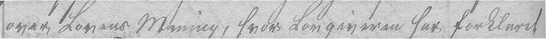over Lovens Mening, hvor Lovgiveren har forklaret
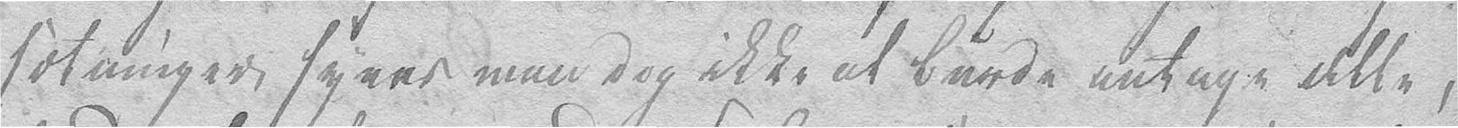sætninger synes man dog ikke at burde antage alle,
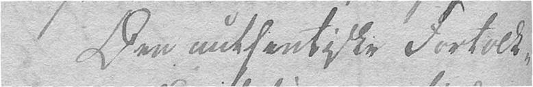Den authentiske Fortolk-
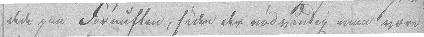dede paa Fornuften, siden der nødvendig maa være
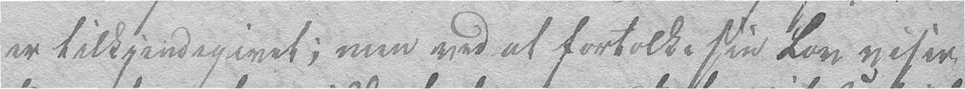er tilkjendegivet; men ved at fortolke sin Lov viser
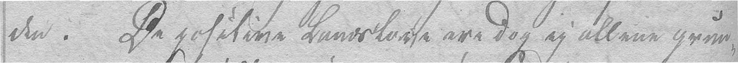den. De positive Landslove ere dog ey allene grun-
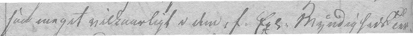saa meget vilkaarligt i dem, f: Exl: MyndighedsTer-
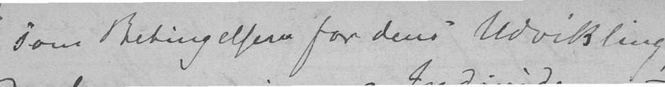som Betingelsen for dens Udvikling,
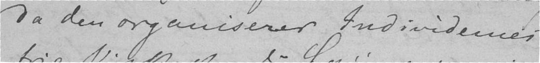da den organiserer Individernes
